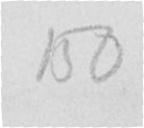150
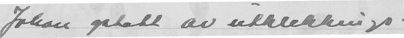Johan optatt av utklekkings-
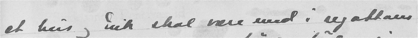et hus og Erik skal være med i regattans
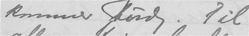kommer lørdag. Til
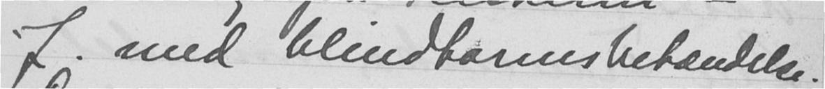J. med blindtarmsbetændelse.
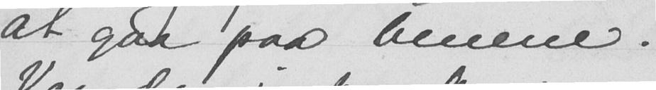at gaa paa benene.
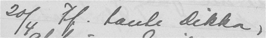20/4 Tf. tante Dikka,
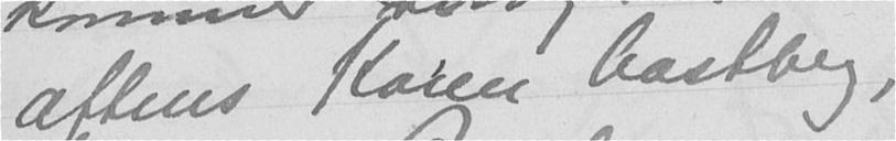aftens Karen Castberg,
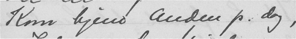Kom hjem anden p. dag,
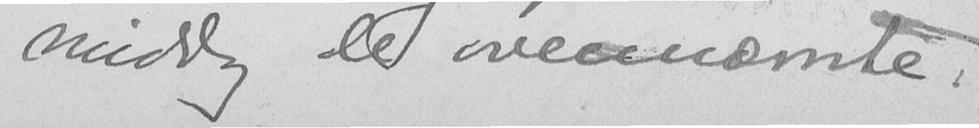middag de ovennævnte.
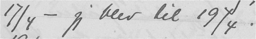17/4 - jeg blev til 19/4.
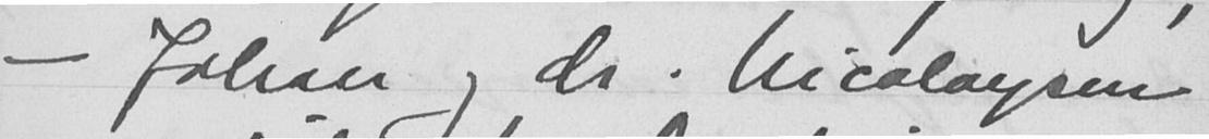- Johan og dr. Nicolaysen
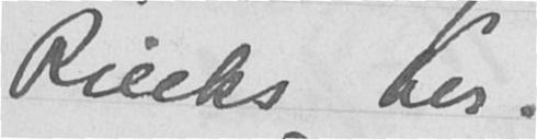Riecks her.
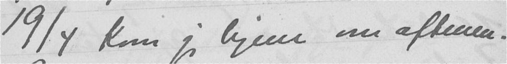19/4 Kom jeg hjem om aftenen.
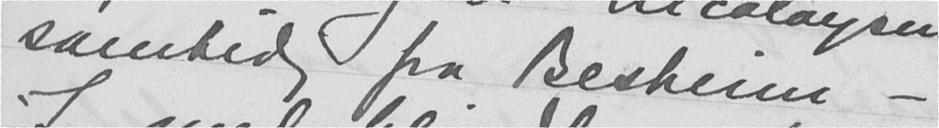samtidig fra Besheim -
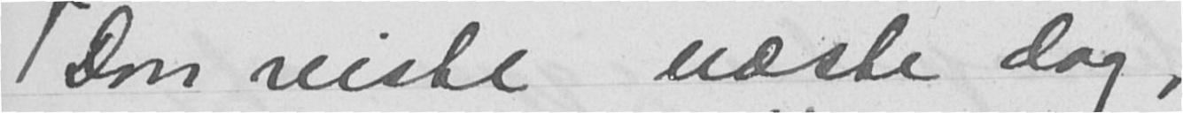Don reiste næste dag,
